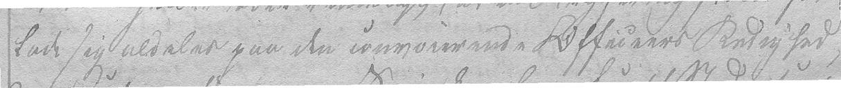lade sig aldeles paa den convoierende Officeers Radighed,
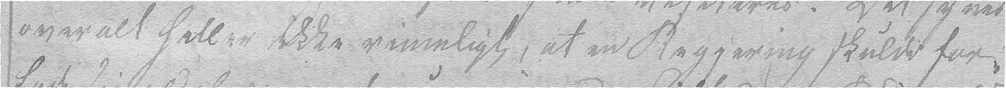overalt heller ikke rimeligt, at en Regjering skulde for-
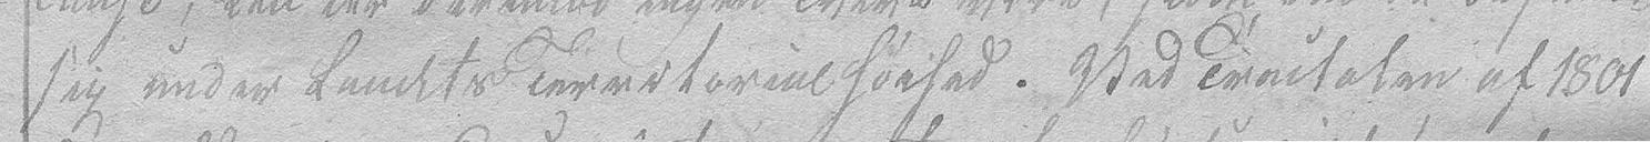sig under Landets Territorialhøihed. Ved Tractaten af 1801
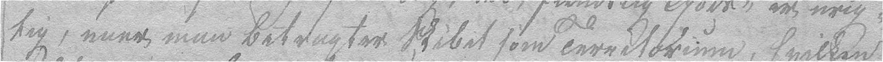tig, naar man betragter Skibet som Territorium, hvilken
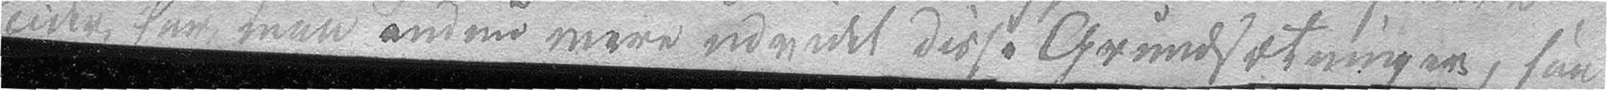Tider har man endnu mere udvidet disse Grundsætninger, saa
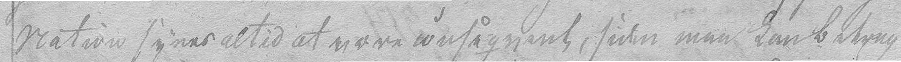Nation synes altid at være conseqvent, siden man kan betrag-
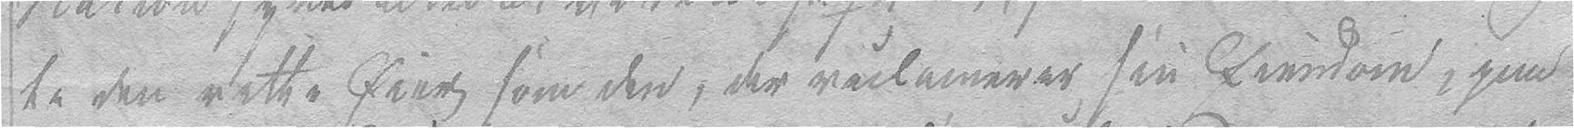te den rette Eier som den, der reclamerer sin Eiendom, paa
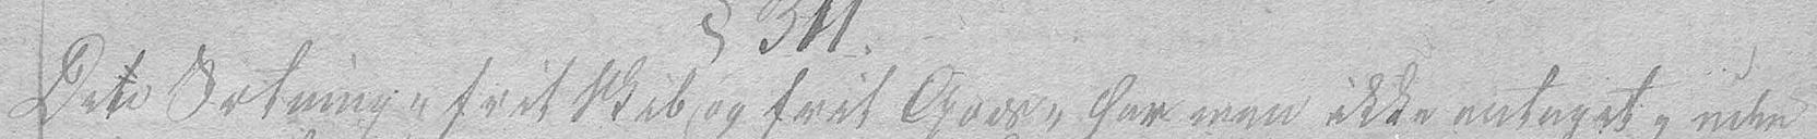Den Sætning "frit Skib og frit Gods" har man ikke antaget, uden
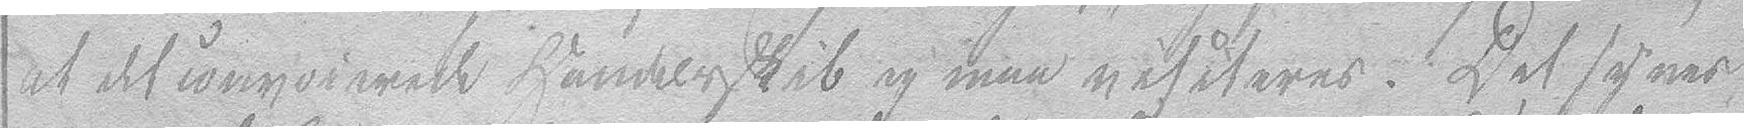at det convoierede Handelsskib ey maa visiteres. Det synes
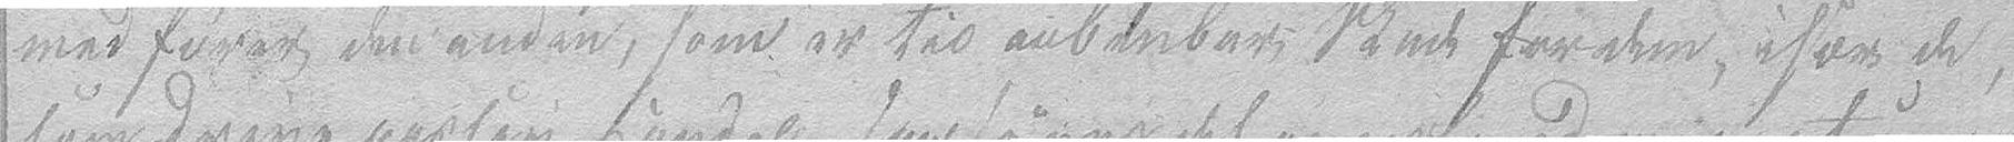medfører den anden, som er til aabenber Skade for dem, især de,
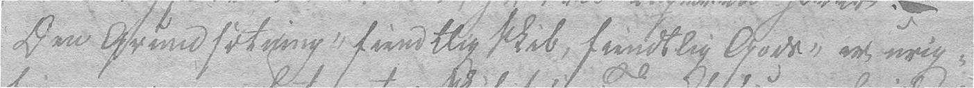Den Grundsætning "fiendtlig Skib, fiendtlig Gods" er urig-
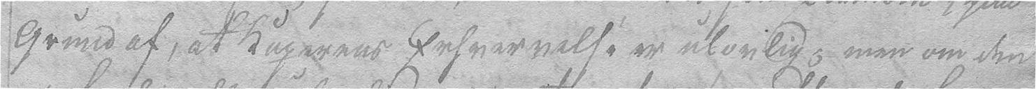Grund af, at Kaperens Erhvervelse er ulovlig; men om den
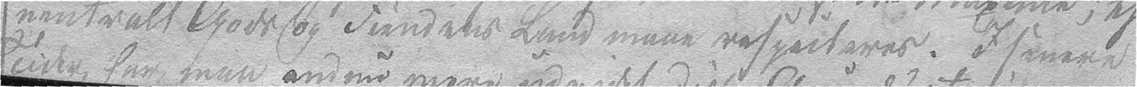neutralt Gods og Fiendens Land maa respecteres. I senere
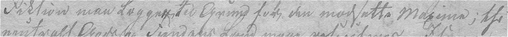Fiktion man lægger til Grund for den modsatte Maxime; thi
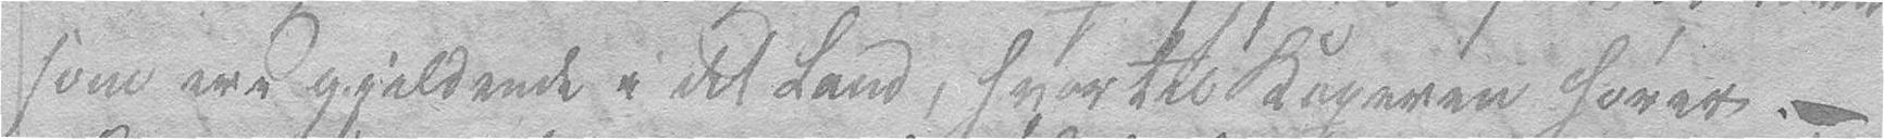som ere gjeldende i det Land, hvortil Kaperen hører. –
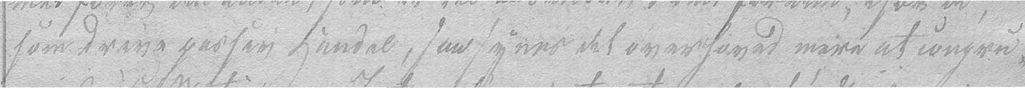som drive passiv Handel, saa synes det overhoved mere at congru-
